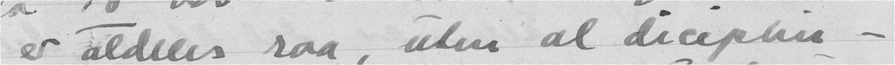er aldeles raa, uten al diciplin -
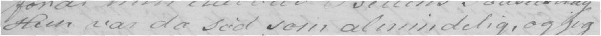Hun var da sød som almindelig, og jeg
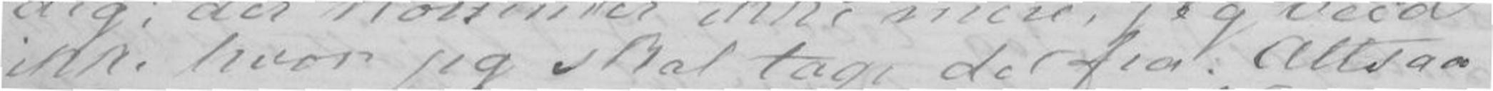ikke hvor jeg skal tage det fra. Altsaa
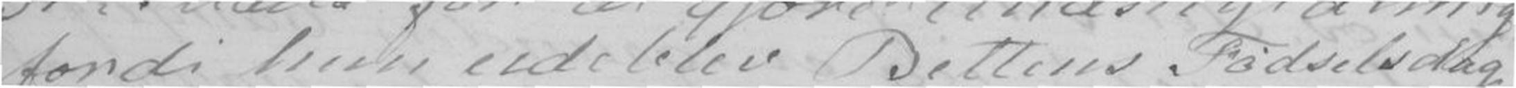fordi hun udeblev i Bettens Fødselsdag.
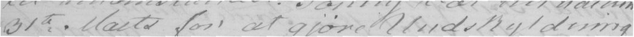31te Marts for at gjøre Undskyldning
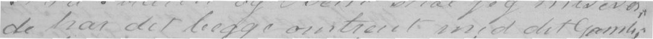de har det begge omtrent med det Gamle,
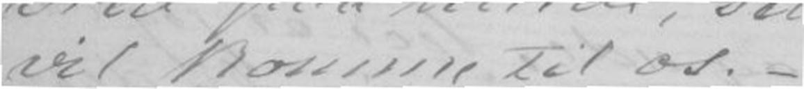vil komme til os. -
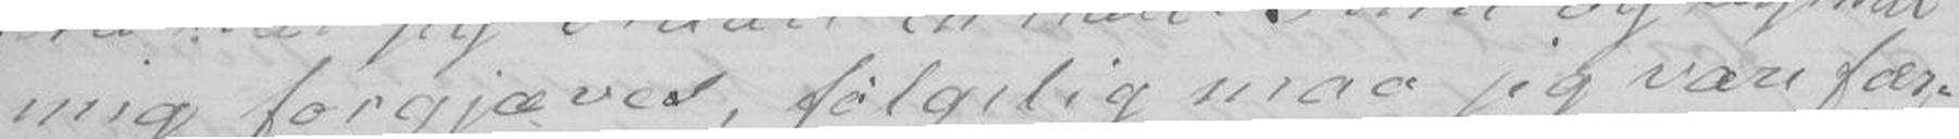mig forgjæves, følgelig maa jeg være fær-
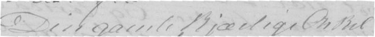Din gamle kjærlige Onkel
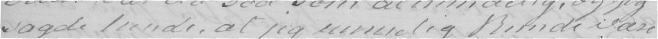sagde hende. at jeg umuelig kunde være
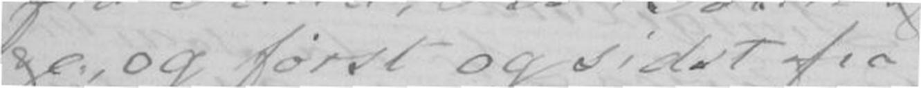ogc., og først og sidst fra
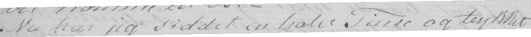Nu har jeg siddet en halv Time og trykket
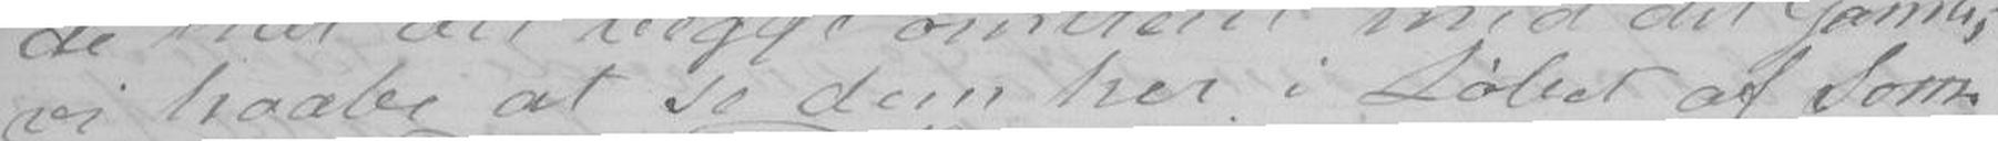vi haabe at se dem her i Løbet af Som-
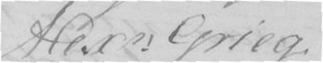Alxr Grieg
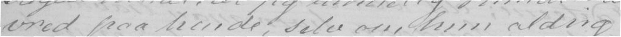vred paa hende, selv om hun aldrig
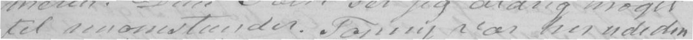til nuomstunder. Tonny var her ude den
